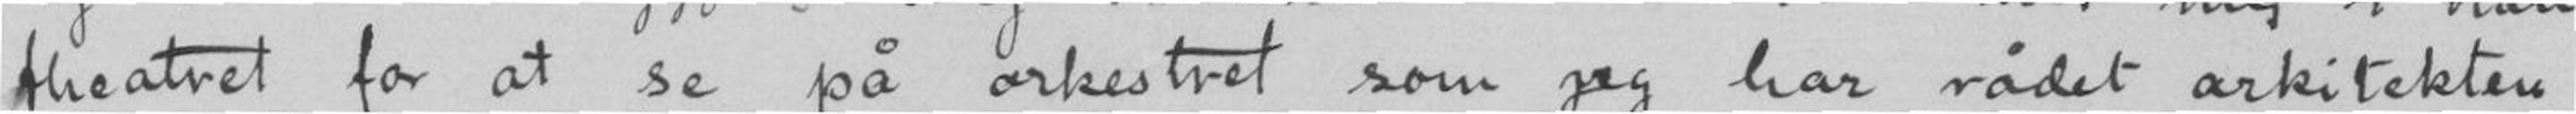theatret for at se på orkestret som jeg har rådet arkitekten
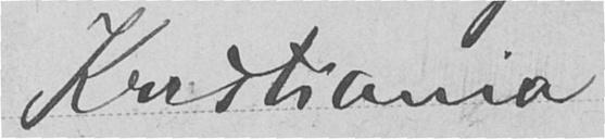Kristiania
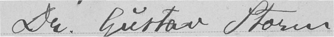Dr. Gustav Storm
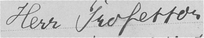Herr Professor
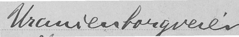Uranienborgveien
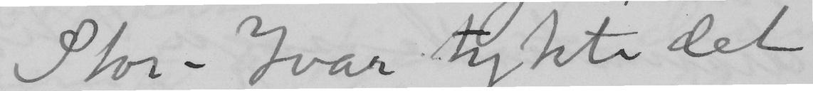Stor-Ivar tykte det
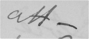att –
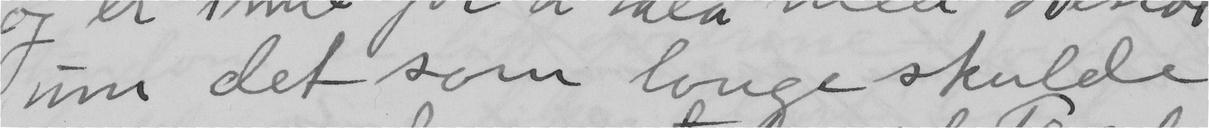um det som lenge skulde
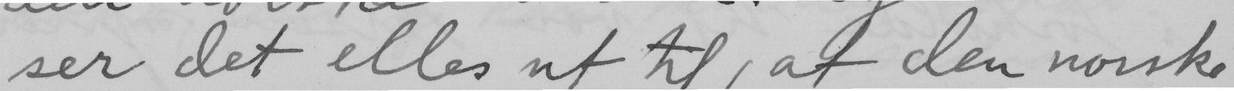ser det elles ut til, at den norske
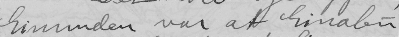Eimunden var at Einaleu
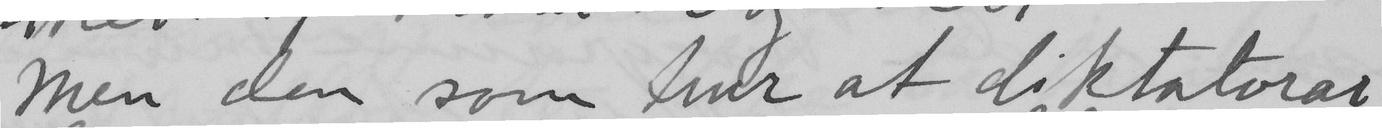Men den som tror at diktatorar
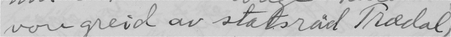vore greid av statsråd Trædal.
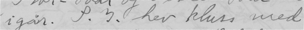igår. S.I. hev kluss med
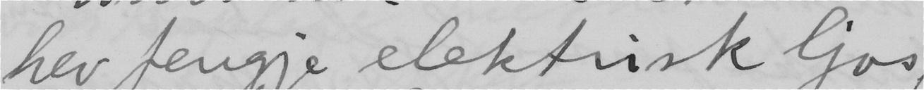hev fengje elektrisk ljos.
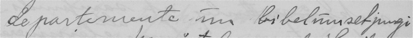departemente um bibelumsetjingi
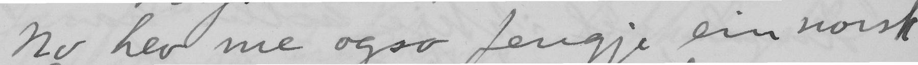No hev me ogso fengje ein norsk
